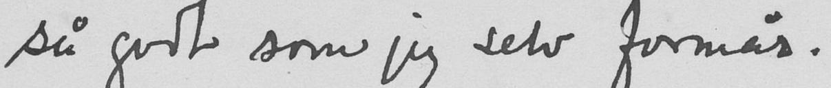så godt som jeg selv formår.
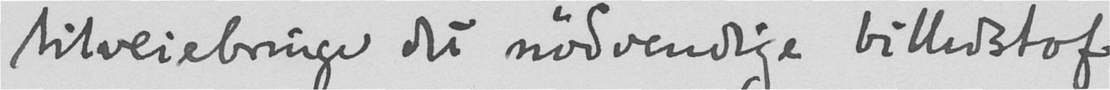tilveiebringe det nødvendige billedstof
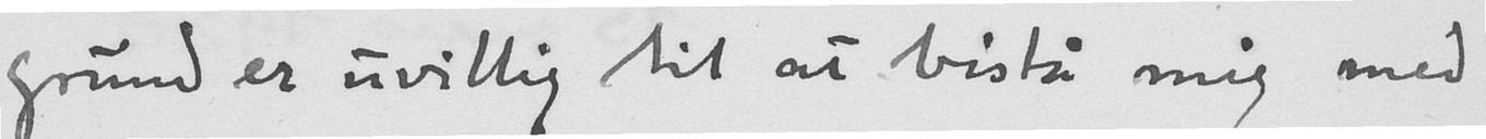grund er uvillig til at bistå mig med
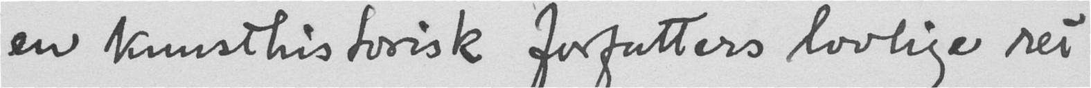en kunsthistorisk forfatters lovlige ret
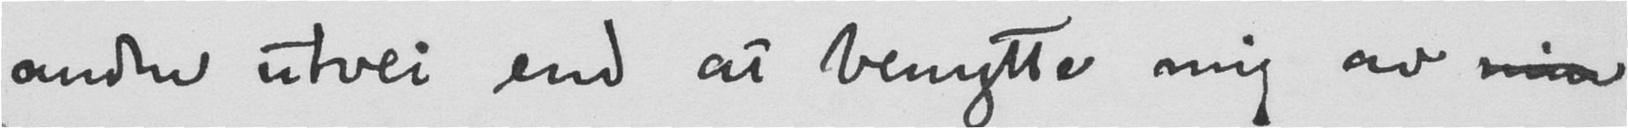anden utvei end at benytte mig av min
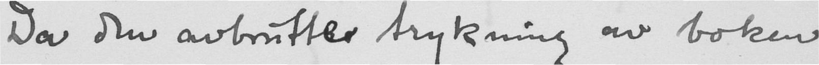Da den avbrutte trykning av boken
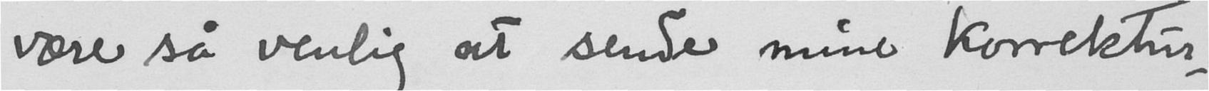være så venlig at sende mine korrektur-
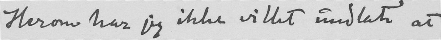Herom har jeg ikke villet undlate at
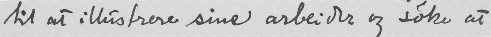til at illustrere sine arbeider og søke at
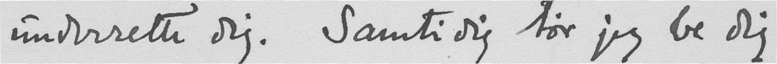underrette dig. Samtidig tør jeg be dig
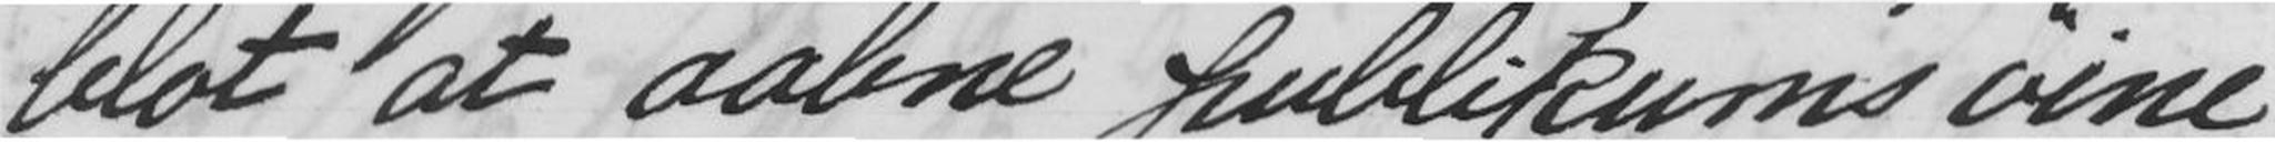blot at aabne publikums øine
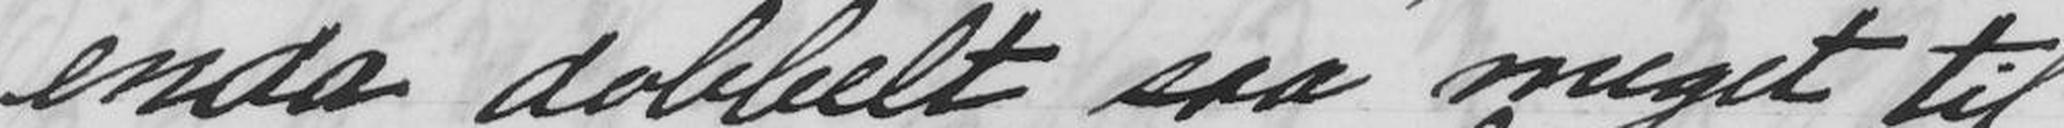enda dobbelt saa meget til,
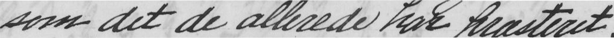som det de allerede har præsteret,
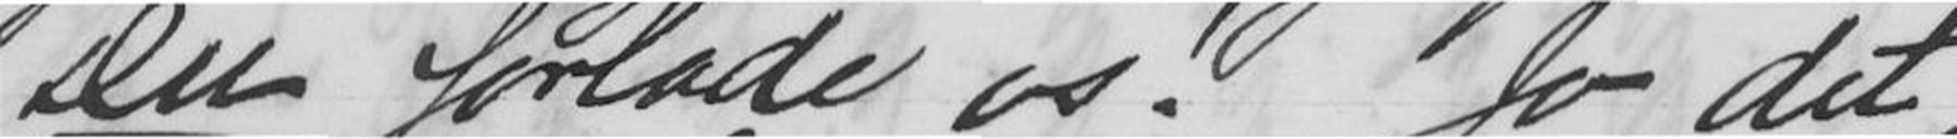Du forlade os! Jo det
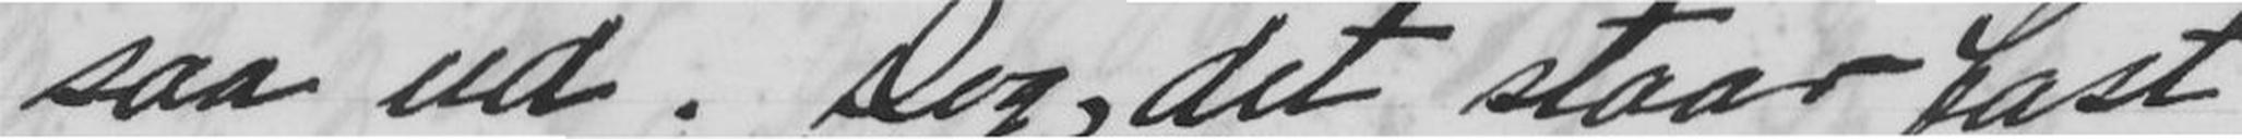saa ud. Dog, det staar fast,
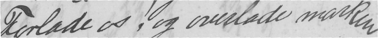Forlade os! og overlade marken
